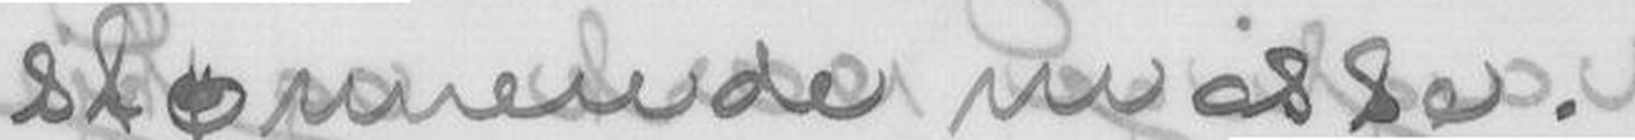stønnende masse.
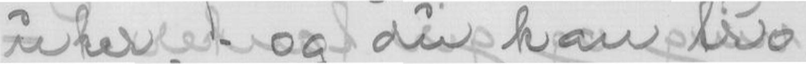uker, - og du kan tro
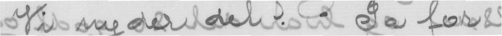Vi nyder det! - Ja for
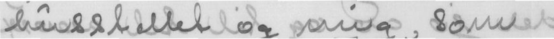husstellet og mig, som
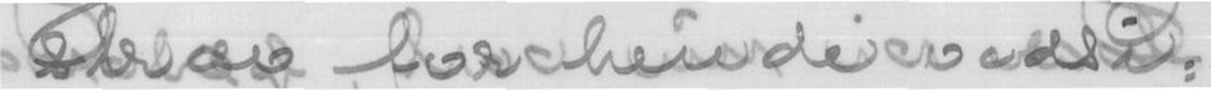stræv for hende vedsi-
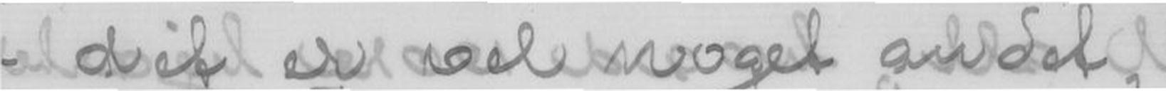det er vel noget andet,
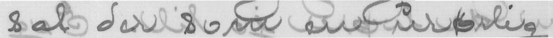sat der som en urørlig
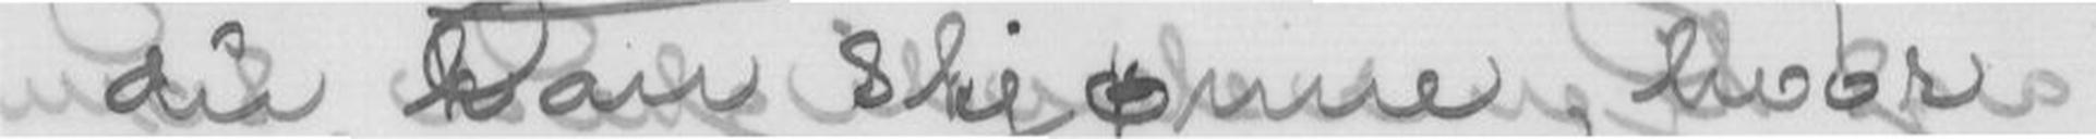du kan skjønne hvor
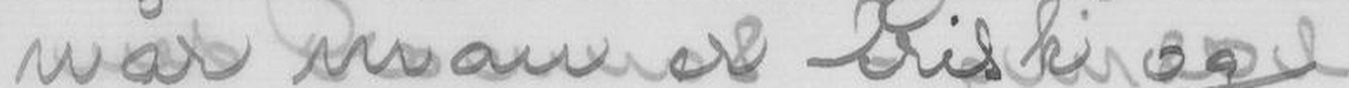når man er frisk og
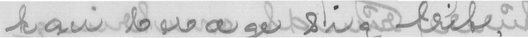kan bevæge sig frit,
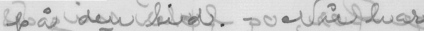på dem tid. - Nu har
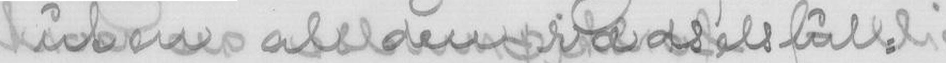uten al den rædselsful-
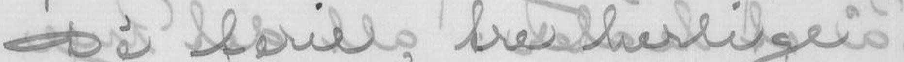Dé ferie, tre herlige
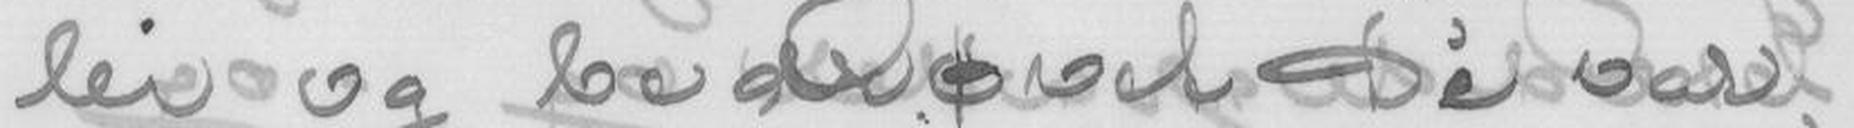lei og bedrøvet Dé var,
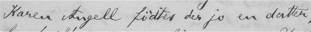Karen Angell fødtes der jo en datter,
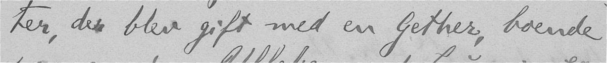ter, der blev gift med en Gether, boende
